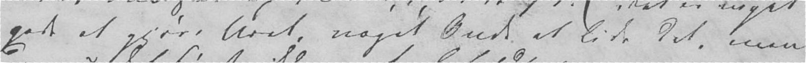godt at gjøre Uret, noget Ondt at lide Det, men
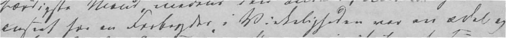anseet for en Forbryder i Virkeligheden var en ædel og
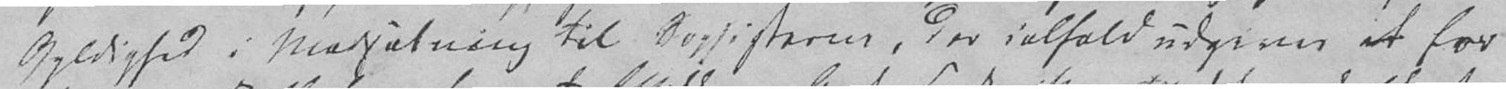Gyldighed i Modsætning til Sophisterne, der ialfald udgives at for
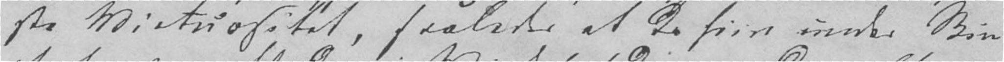ste Virtuositet, saaledes at de hiin under Skin
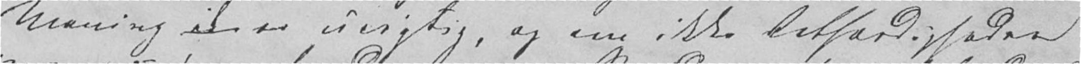Mening ik er urigtig, og om ikke Retfærdigheden
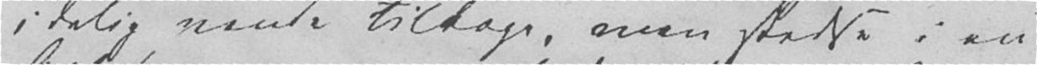idelig vende tilbage, men stedse i en
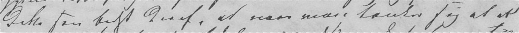Dette sees bedst deraf, at naar man tænker sig at et
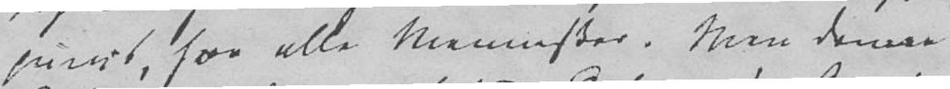punct, for alle Mennesker. Men denne
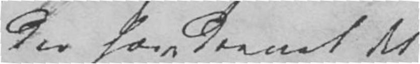der have drevet det
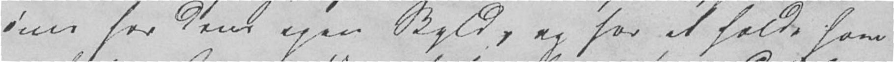øves for dens egen Skyld, og for at holde ham
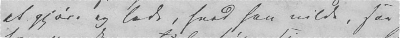at gjøre og lade, hvad han vilde, saa
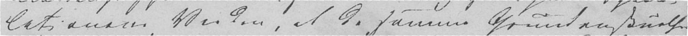lationens Verden, at de samme Grundanskuelser
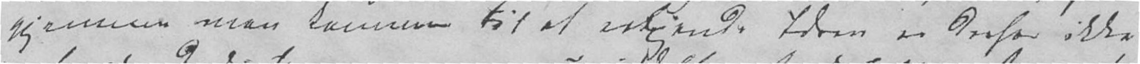gjennem man kommer til at erkjende Ideen er derfor ikke
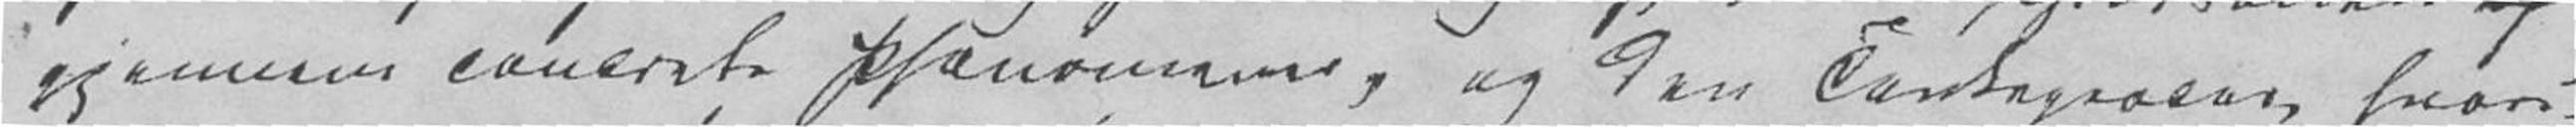gjennem concrete Phænomener, og den Tænkeproces, hvori-
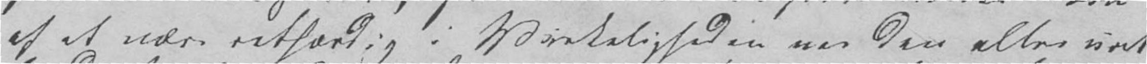af at være retfærdig i Virkeligheden var den aller uret-
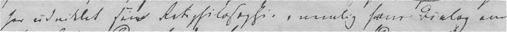har udviklet sin Retsphilosophie, nemlig som Dialog om
